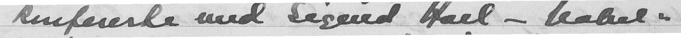konfererte med Sigurd Hoel - nobel-
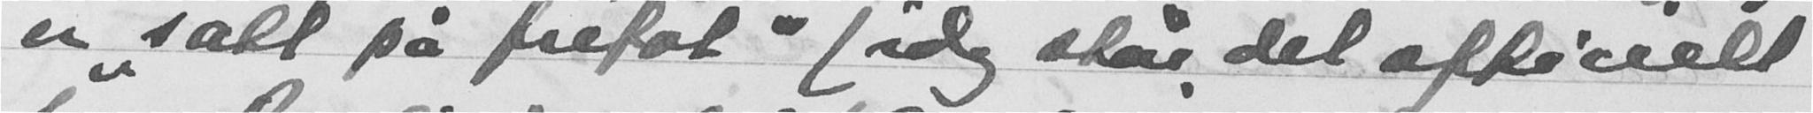er "satt på frifot" (idag står det officielt
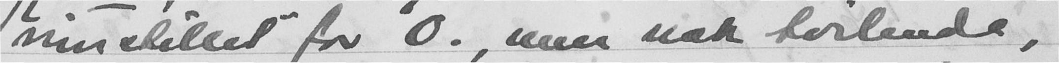innstillet for O., men nok tvilende,
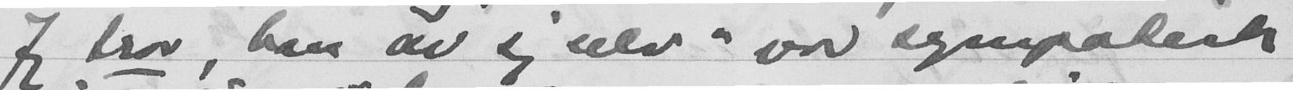Jeg tror, han "av sig selv" var sympatisk
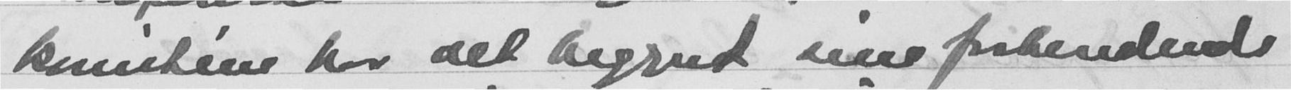komitiéen har alt begynd sine forberedende
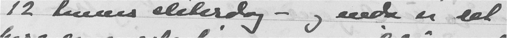12 timers sliterdag - og enda er det
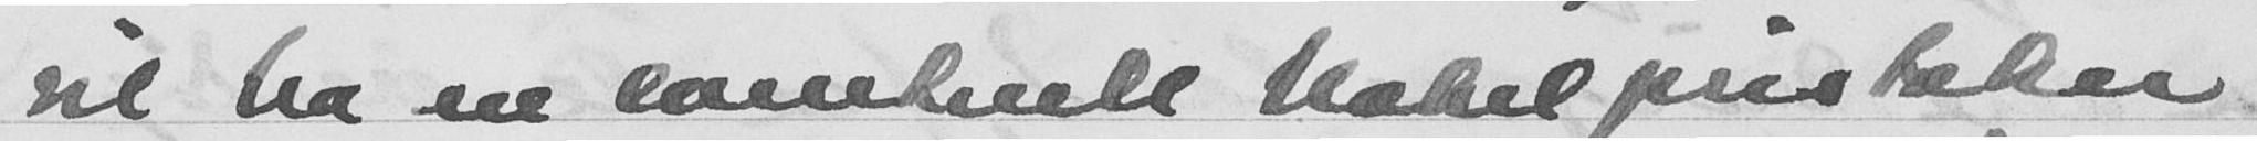vil ha en samlende Nakelpristæker
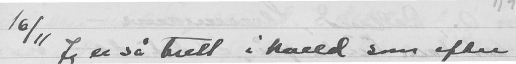16/11 Jeg er så trøtt i kveld som efter
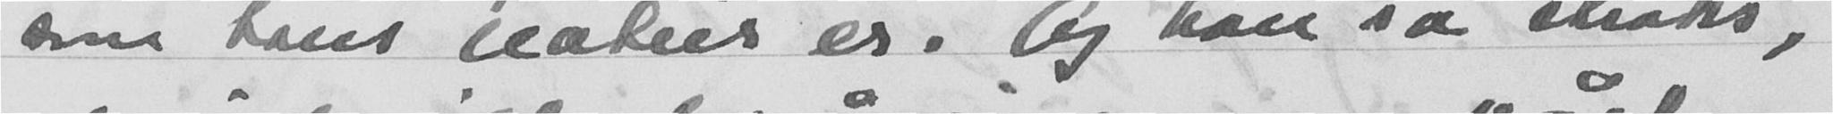som hans natur er. Og han sa straks,
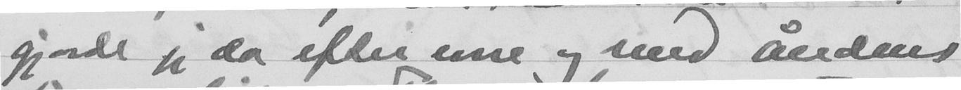gjorde jeg da efter evne og med åndens
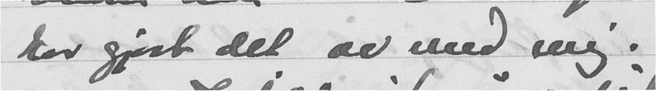har gjort det av med mig.
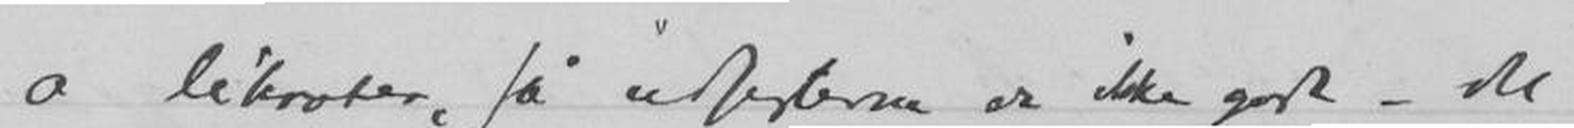o libroter så udsigterne er ikke gode - det
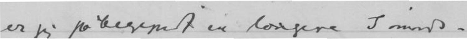er jeg påbegyndt en længere I minds-
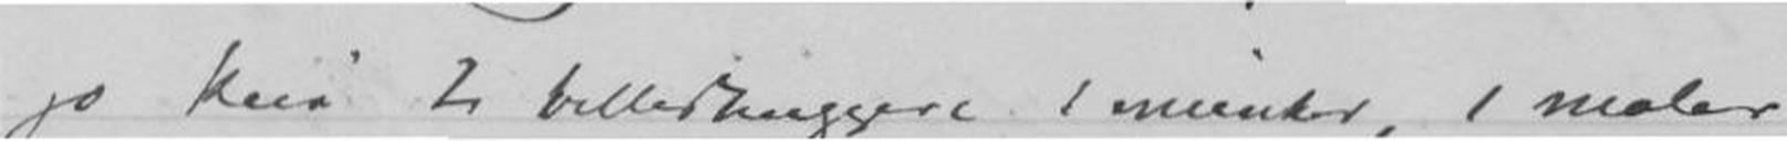jo kun to billedshuggere 1 musiker, 1 maler
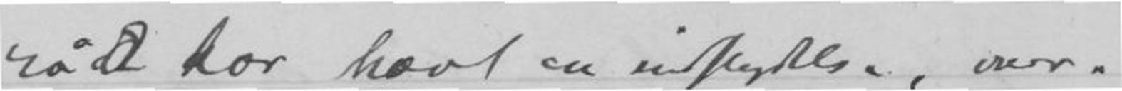råd har havt en indflydelse, over-
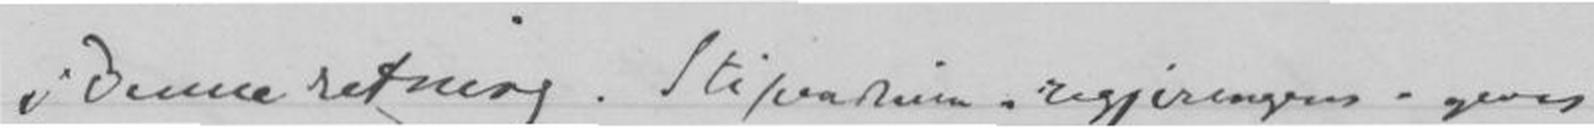i denne retning. Stipendium - regjeringens - gives
